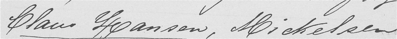Claus Hansen, Mickelsen
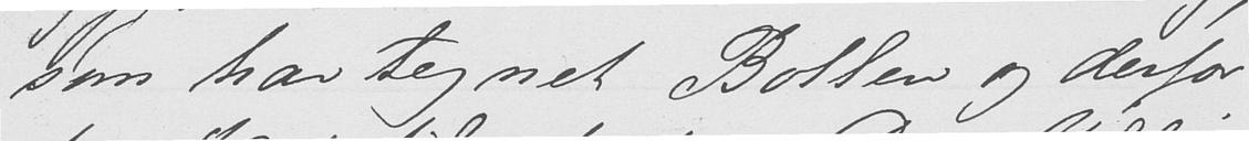som har tegnet Bollen og derfor
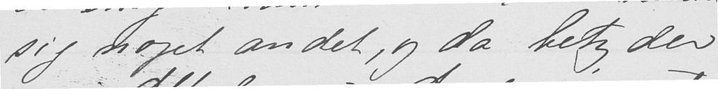sig noget andet, og da betyder
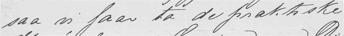saa vi faar ta de praktiske
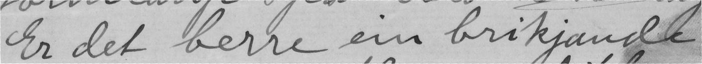Er det berre en brikjande
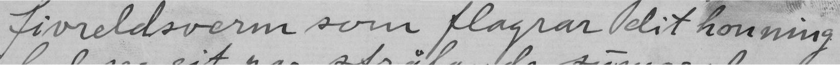fivreldsverm som flagrar dit honning
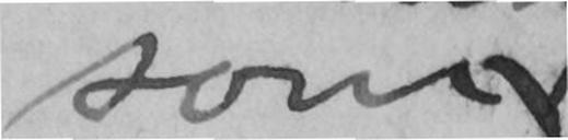som
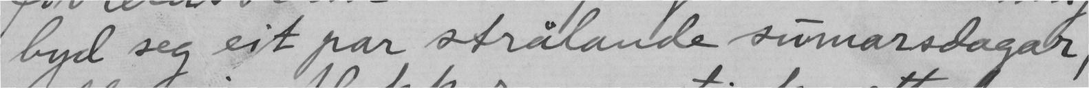byd sig eit par strålande sumarsdagar,
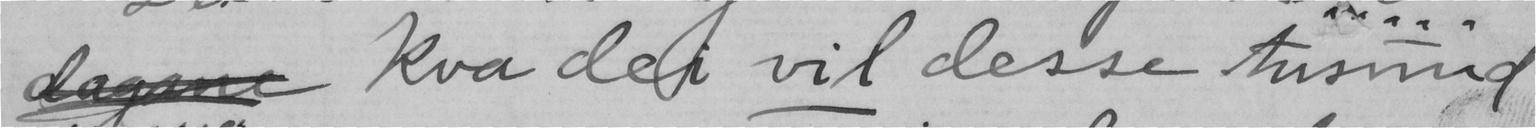dagene kva dei vil desse tusind
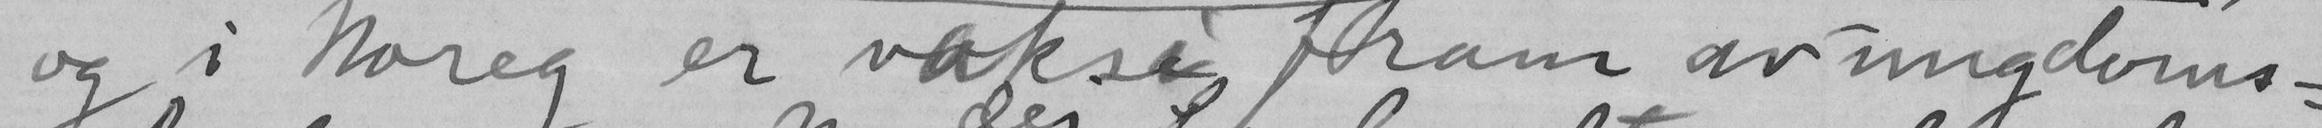og i Noreg er vakse fram av ungdoms-
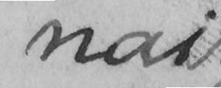naivt
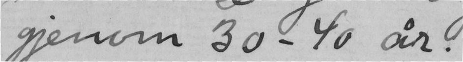gjenom 30-40 år.
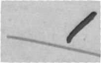1
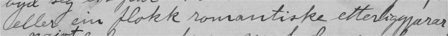eller ein flokk romantiske etterliggjarar,
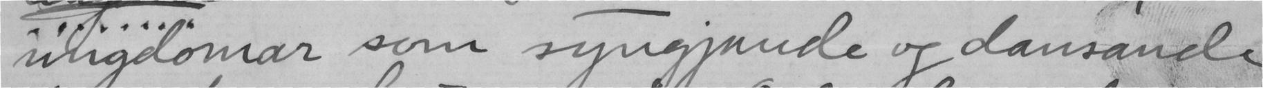ungdomar som syngjande og dansande
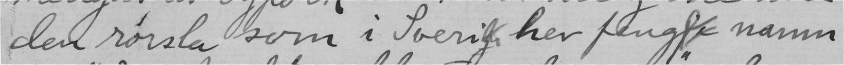den rørsla som i Sverige her fengje namn
